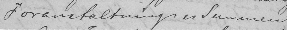Foranstaltning er Summen
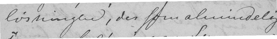løsningen, der som ualmindelig
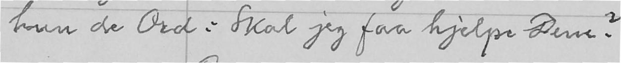hun de Ord: Skal jeg faa hjelpe Dem?
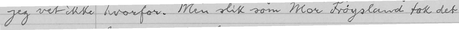jeg vet ikke hvorfor. Men slik som Mor Frøysland tok det
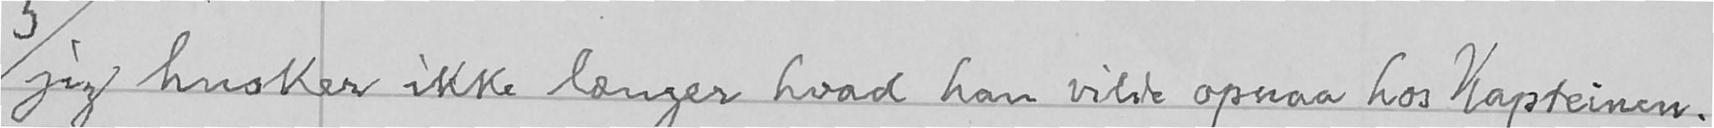jeg husker ikke længer hvad han vilde opnaa hos Kapteinen.
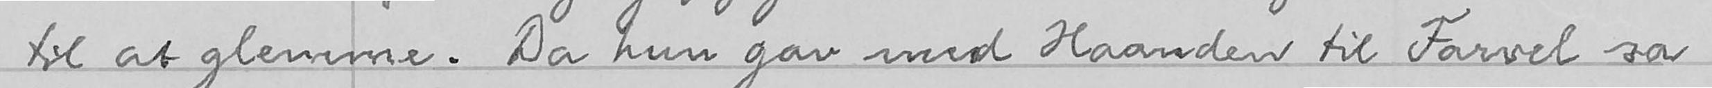til at glemme. Da hun gav med Haanden til Farvel sa
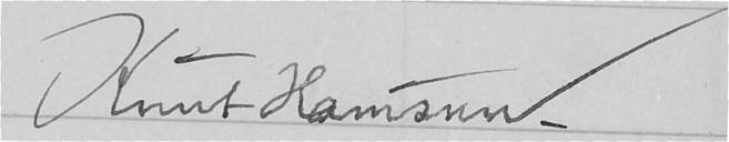Knut Hamsun.
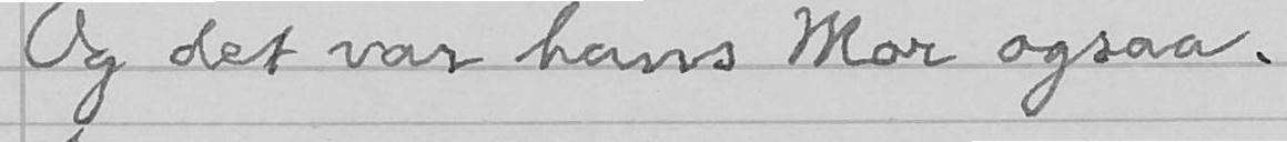Og det var hans Mor ogsaa.
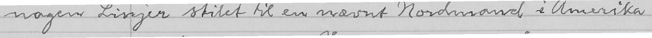nogen Linjer stilet til en nævnt Nordmand i Amerika
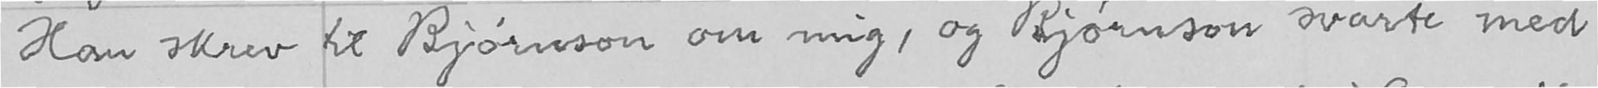Han skrev til Bjørnson om mig, og Bjørnson svarte med
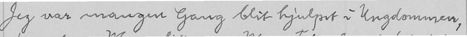Jeg var mangen Gang blit hjulpet i Ungdommen,
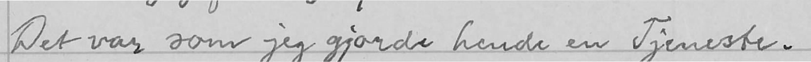Det var som jeg gjorde hende en Tjeneste.
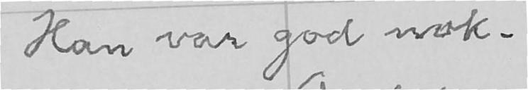Han var god nok.
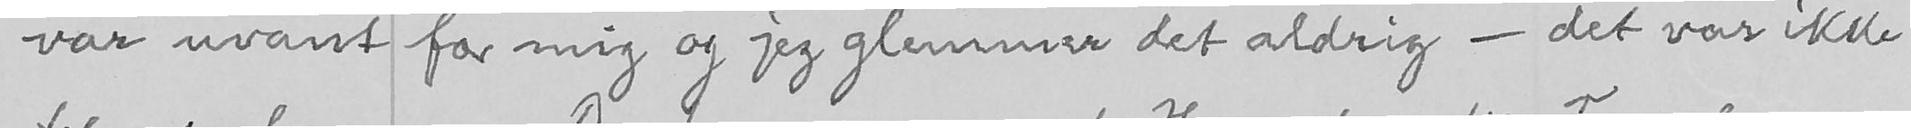var uvant for mig og jeg glemmer det aldrig - det var ikke
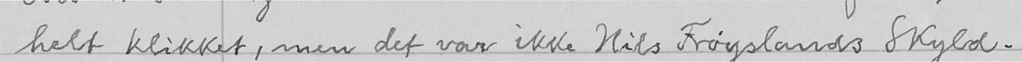helt klikket, men det var ikke Nils Frøyslands Skyld.
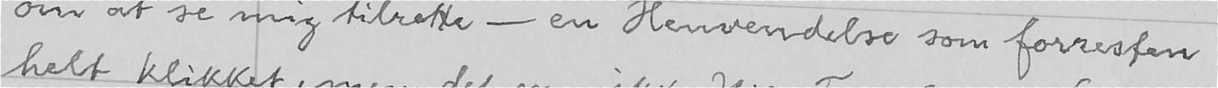om at se mig tilrette - en Henvendelse som forresten
